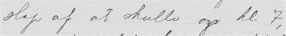slap of at skulle op kl. 7,
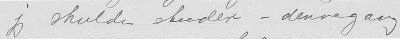jeg skulde studere – dennegang
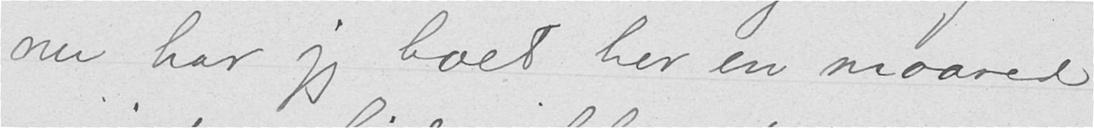nu har jeg boet her en maaned
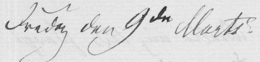Fredag den 9 Marts.
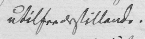utilfredsstillende.
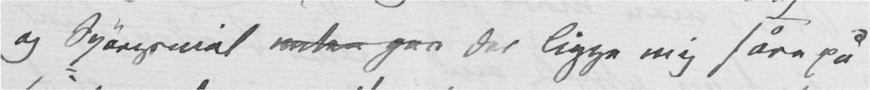og Spørgsmål enten gav der ligge mig såre på
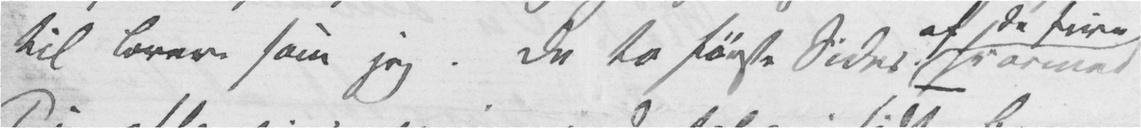til Breve som jeg. De to første Sider, hvormed
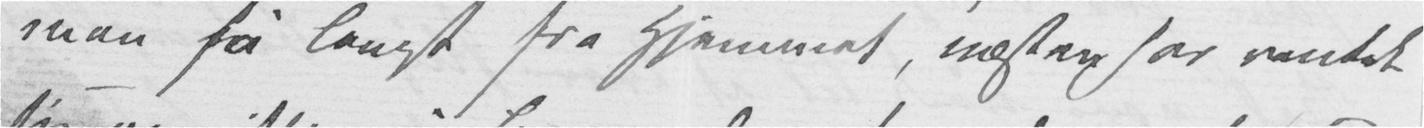man så langt fra Hjemmet, næsten har ventet
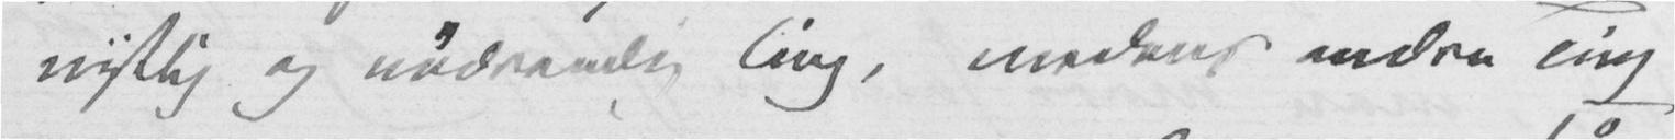nyttig og nødvendig Ting, medens andre Ting
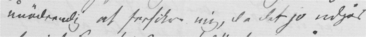unødvendig at forsikre mig, da det jo udgjør
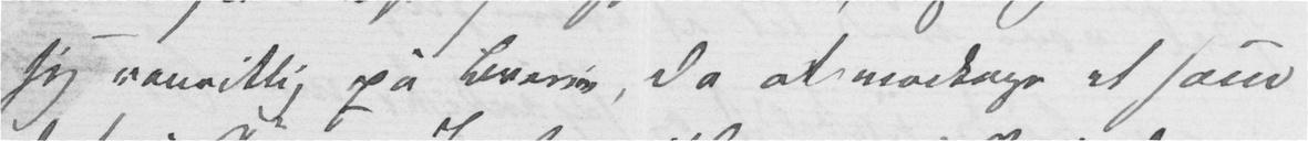sig vanvittig på Breve, da at modtage et som
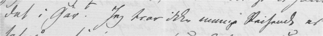det i Går. Jeg tror ikke mange Reisende er
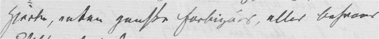Hjerte, enten ganske forbigåes, eller besvares
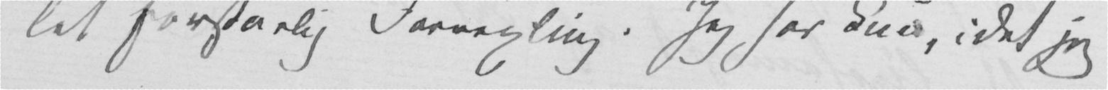let forståelig Forvexling. Jeg har kun, idet jeg
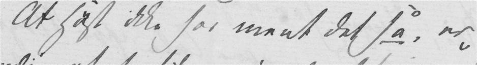At Høst ikke har ment det så, er
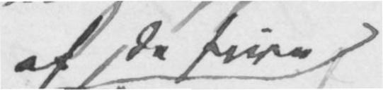af de fire
